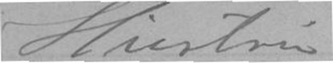Hustru
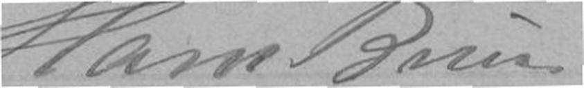Hans Brun
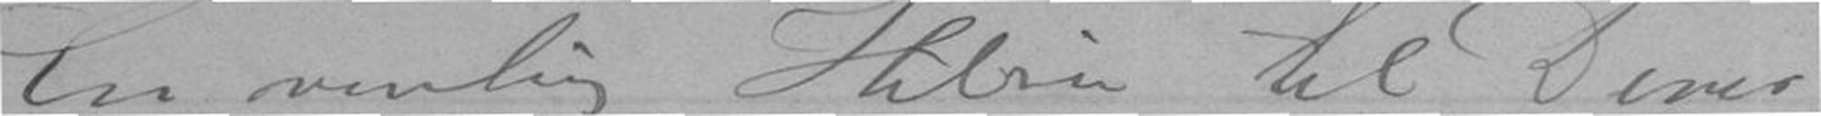En venlig Hilsen til Deres
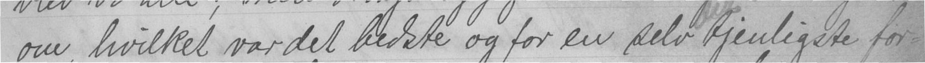om, hvilket var det bedste og for en selv tjenligste for-
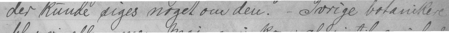der kunde siges noget om den." - Ivrige botanikere
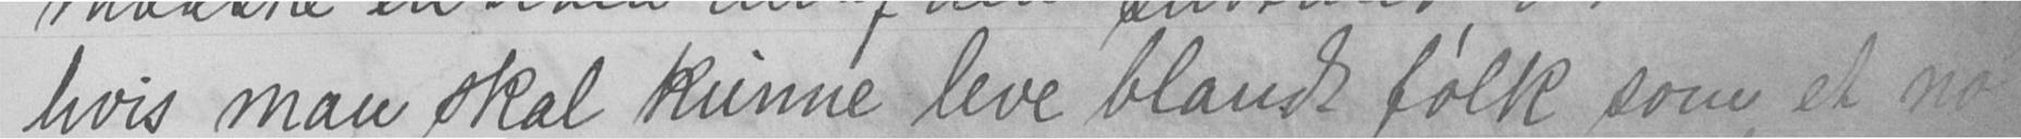hvis man skal kunne leve blandt folk som, et no-
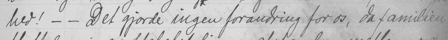hed! - - Det gjorde ingen forandring for os, da familien
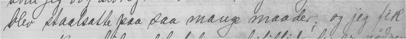blev staalsatte paa saa mange maader; og jeg fik
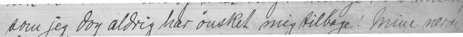som jeg dog aldrig har ønsket mig tilbage! mine nerver
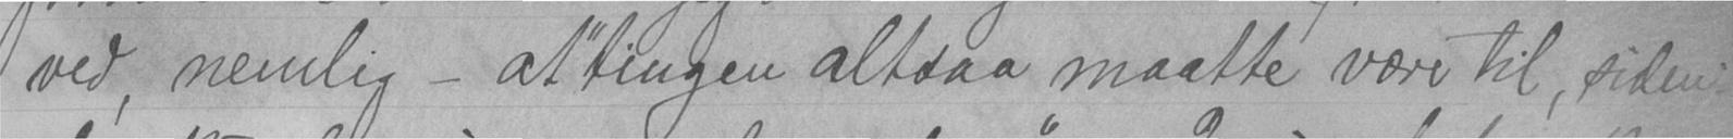ved, nemlig - at "tingen altsaa maatte være til, siden
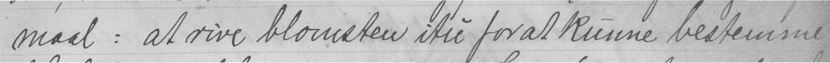maal: at rive blomsten itu for at kunne bestemme
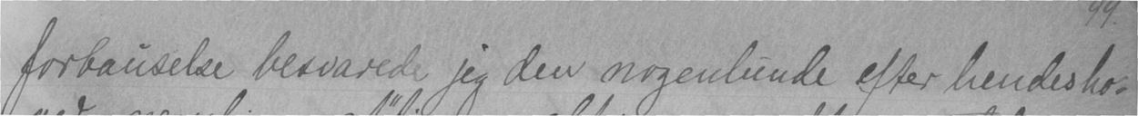forbauselse besvarede jeg den nogenlunde efter hendes ho-
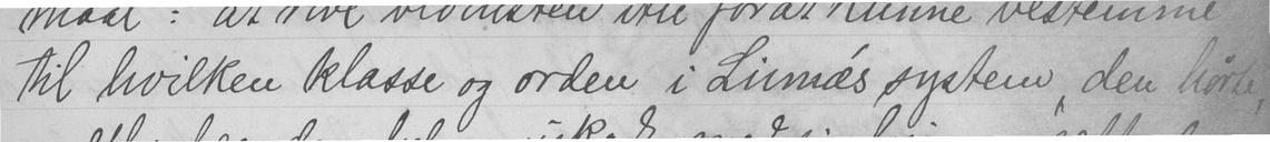thil hvilken klasse og orden i Linnés system, den hørte,
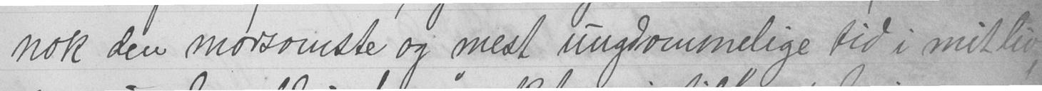nok den morsomste og mest ungdommelige tid i mit liv,
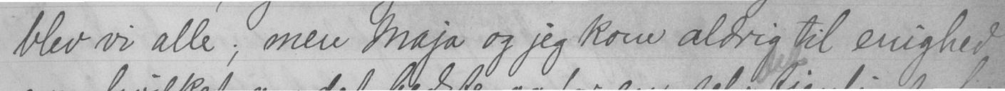blev vi alle; men Maja og jeg kom aldrig til enighed
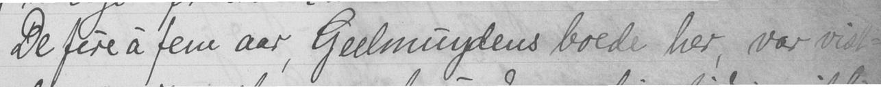De fire à fem aar, Geelmuydens boede her, var vist-
